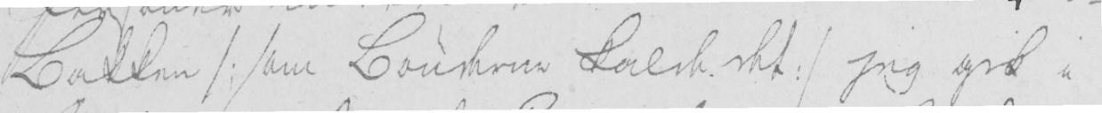Bakken /: som Bønderne kalde det :/ jeg gik i
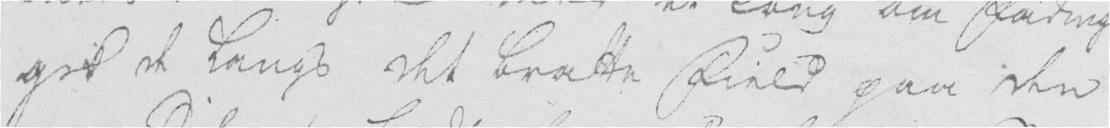gik de langs det bratte Field paa den
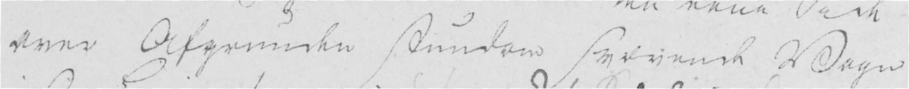over Afgrunden stundom svævende Vogn
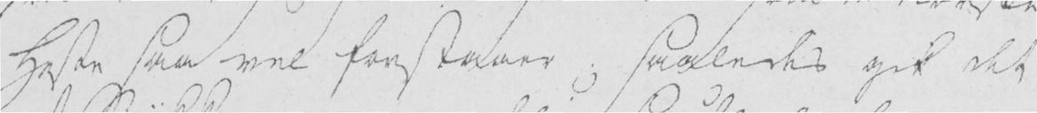Heste saa vel forstaaer; saaledes gik det
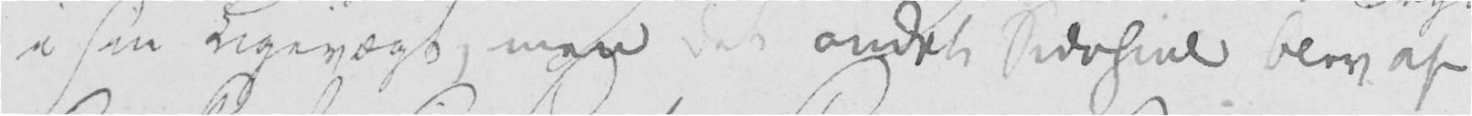i sin Ligevægt, men det andet Sidehiul blev af
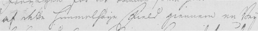af dette himmelhøye Field giennem en Vey
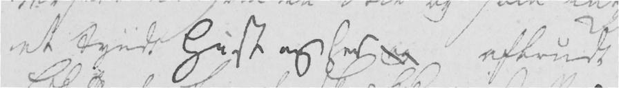et tyndt Hist og her  afbrudt
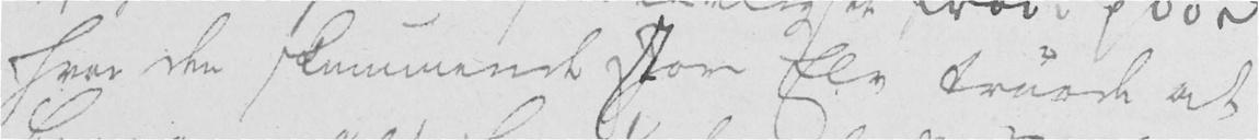hvor den skummende store Elv truede at
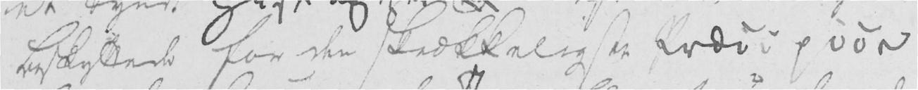beskyttende for den skrækkeligste præcipoor
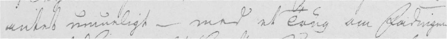intet umueligt - med et Taug om Fodringen
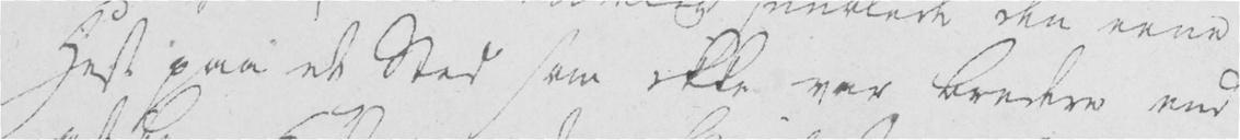Hest paa et Sted som ikke var bredere end
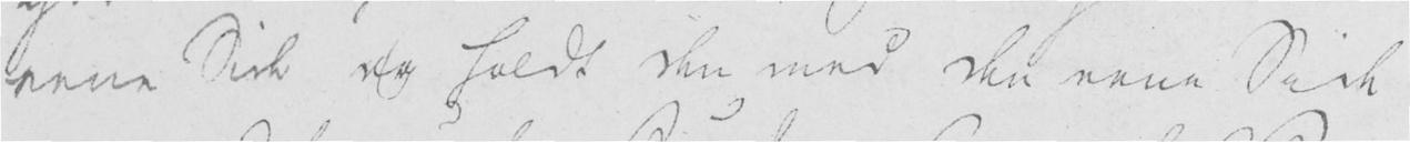eene Side og holdt den med den eene Side
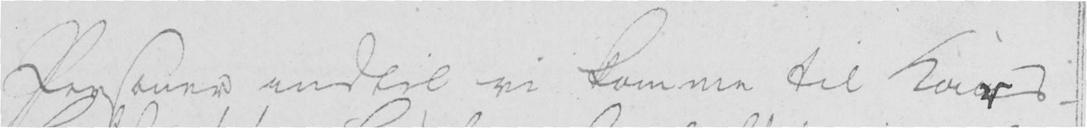Personer indtil vi komme til Nord-
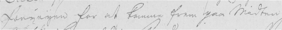forveyen for at komme frem paa Midten
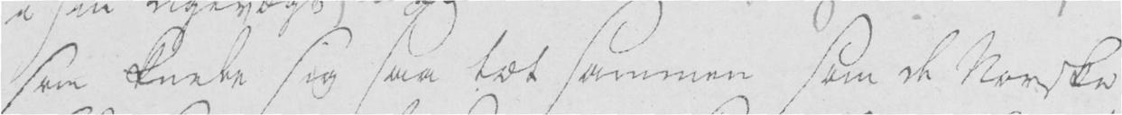som kuede sig saa tæt sammen som de Norske
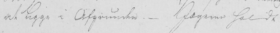at ligge i Afgrunden. - Jægeren holdt
